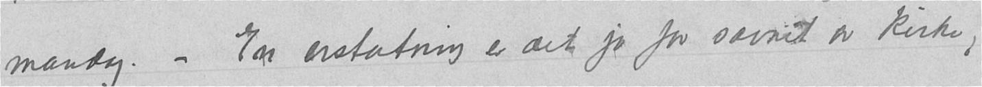mandag. – En erstatning er det jo for savnet av kirke,
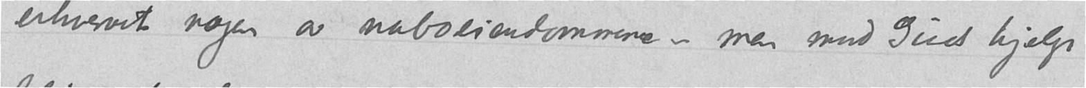erhvervet nogen av naboeiendommene – men med Guds hjelp
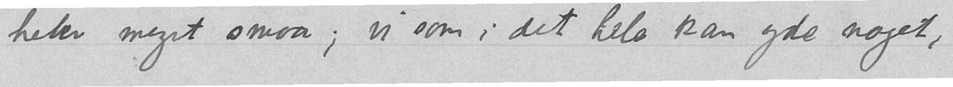heter meget smaa; vi som i det hele kan yde noget,
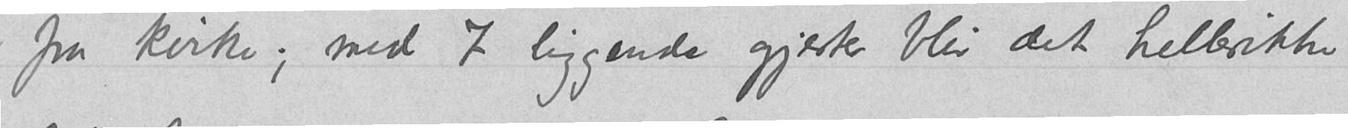fra kirke; med 7 liggende gjester blir det hellerikke
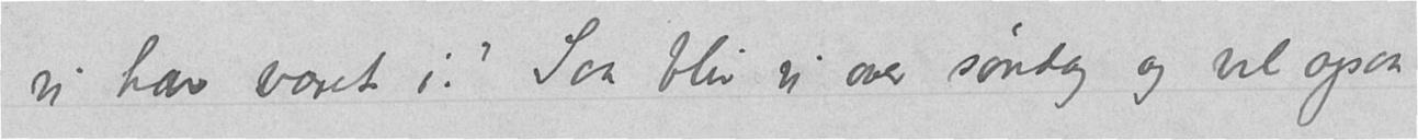vi har været i." Saa blir vi over søndag og vel ogsaa
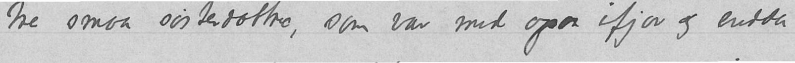tre smaa søsterdøttre, som var med ogsaa ifjor og endda
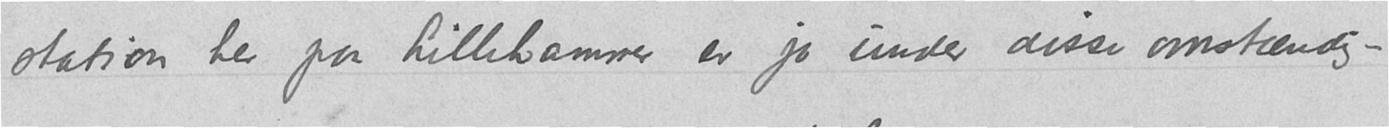station her paa Lillehammer er jo under disse omstændig-
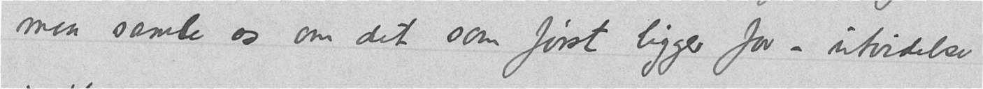maa samle os om det som først ligger for – utvidelse
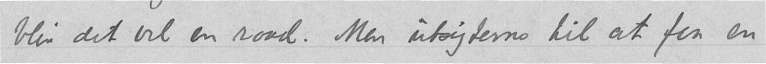blir det vel en raad. Men utsigterne til at faa en
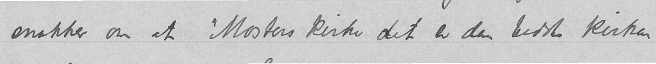snakker om at «Mosters kirke det er den bedste kirken
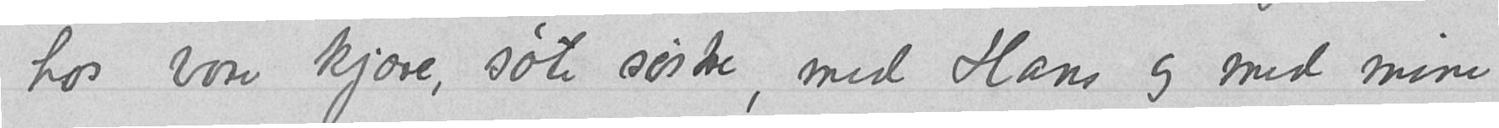hos vore kjære, søte søstre, med Hans og med mine
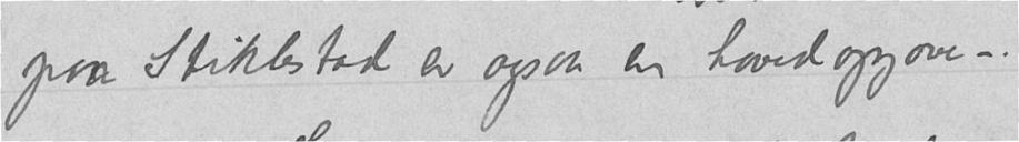paa Stiklestad er ogsaa en hovedopgave –.
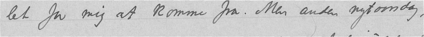let for mig at komme fra. Men anden nytaarsdag,
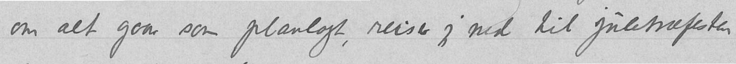om alt gaar som planlagt, reiser jeg ned til juletræfesten
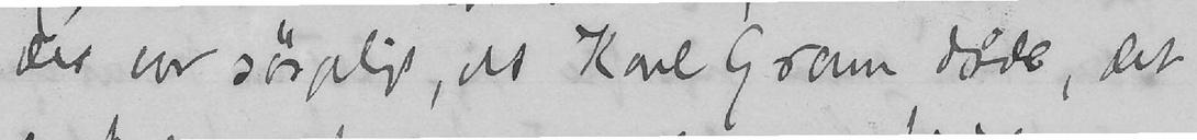Det var sørgeligt, at Karl Gram døde, det
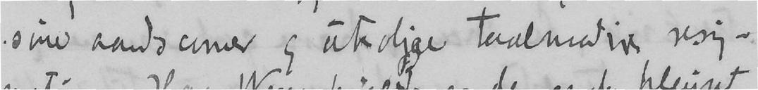sine aandsevner og utrolige taalmodig resig-
